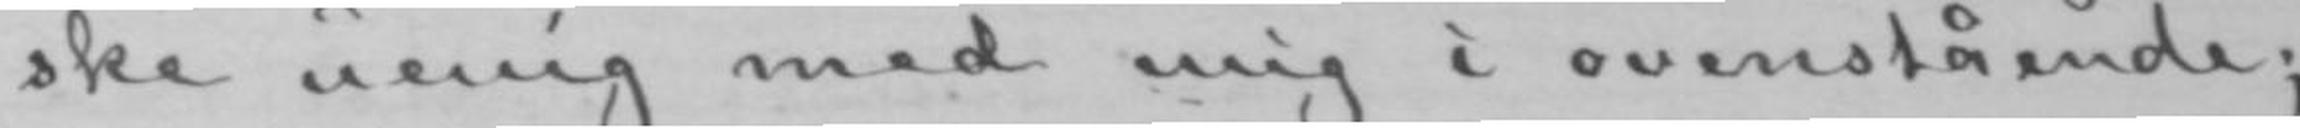ske uenig med mig i ovenstående;
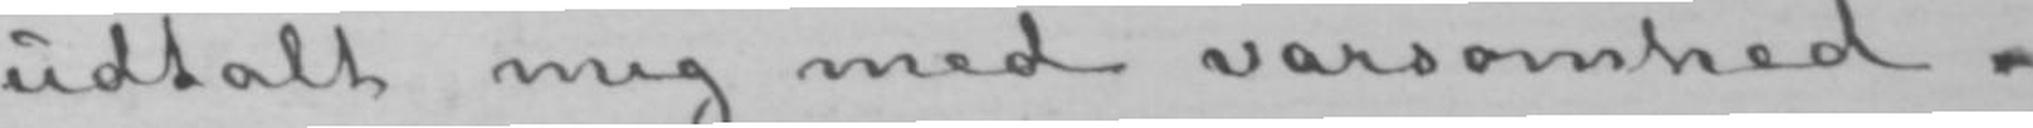udtalt mig med varsomhed.
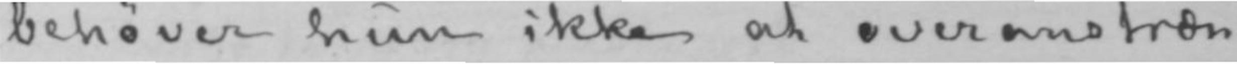behøver hun ikke at overanstræn-
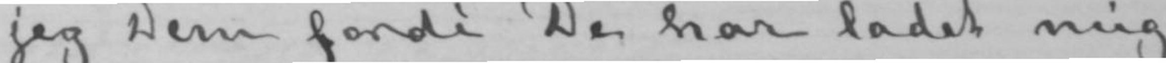jeg Dem fordi De har ladet mig
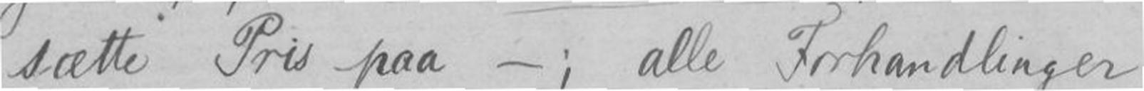sætte Pris paa - i alle Forhandlinger
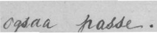ogsaa passe.
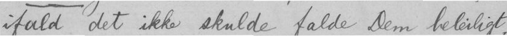ifald det ikke skulde falde Dem beleiligt,
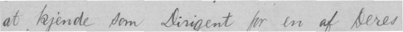at kjende som Dirigent for en af Deres
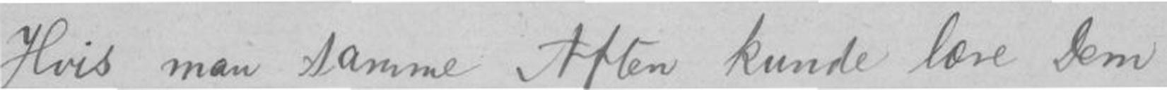Hvis man samme Aften kunde lære Dem
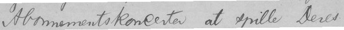Abonnementskoncerter at spille Deres
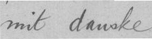mit danske
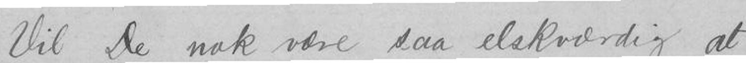Vil De nok være saa elskværdig at
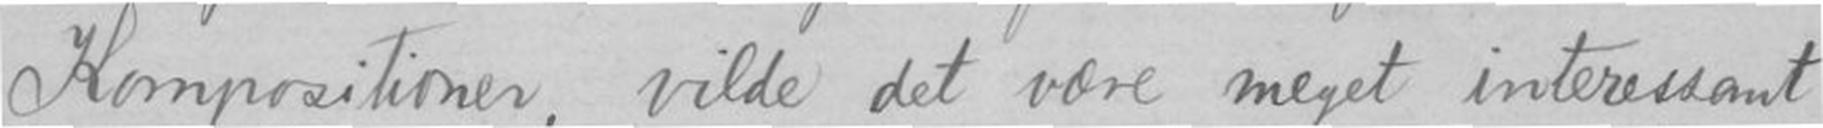Kompositioner, vilde det være meget interessant
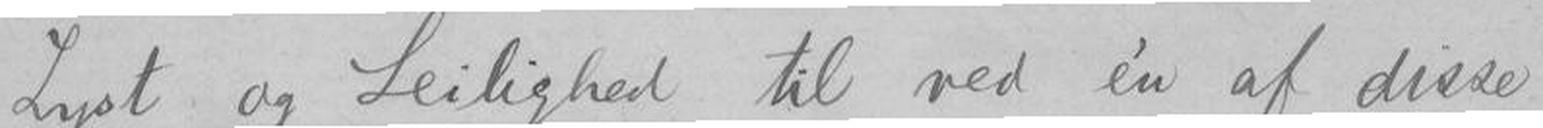Lyst og Leilighed til ved én af disse
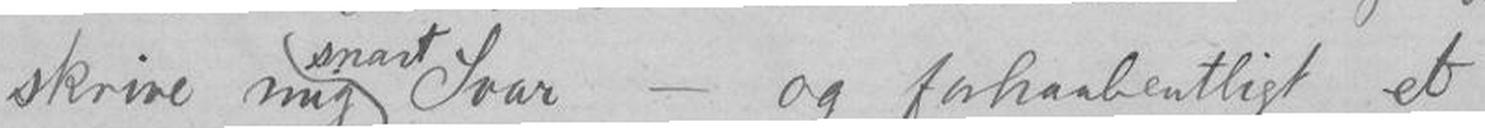skrive mig Svar - og forhaabentligt et
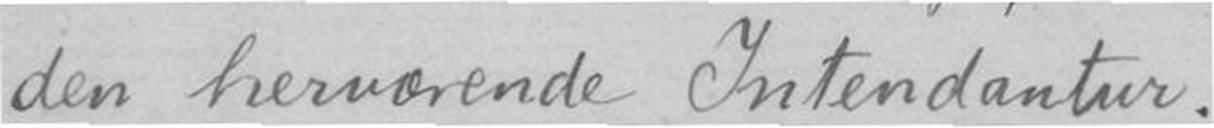den herværende Intendantur.-
